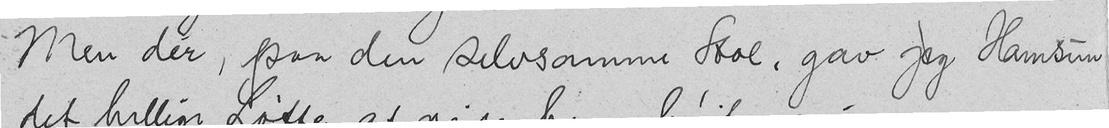Men der, paa den selvsamme Stol, gav jeg Hamsun
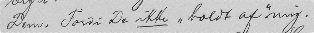Dem. Fordi De ikke "holdt af" mig.
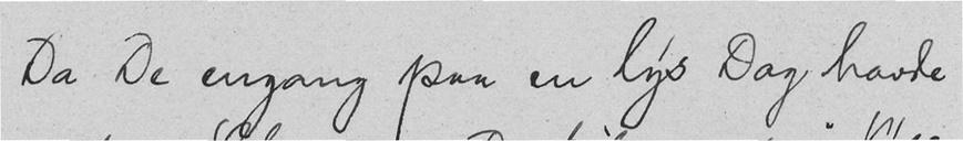Da De engang paa en lys Dag havde
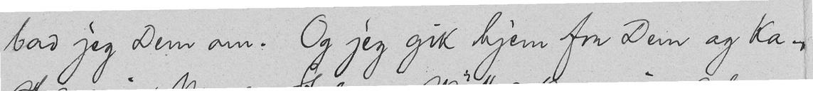bad jeg Dem om. Og jeg gik hjem fra Dem og ka-
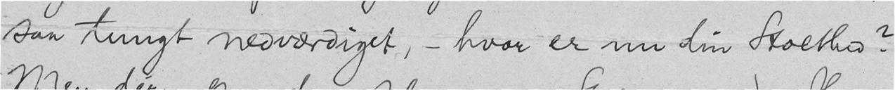saa tungt nedværdiget, - hvor er nu din Stolthed?
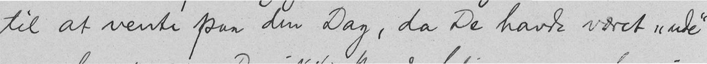til at vente paa den Dag, da De havde været "ude"
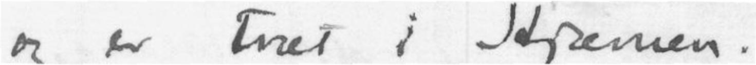og er træt i Hjærnen.
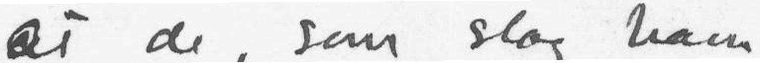at de, som slog ham
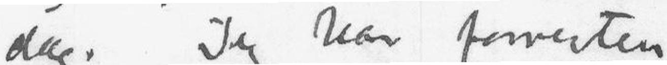dag. Jeg har forresten
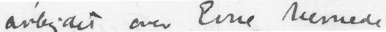arbejdet over Evne hernede
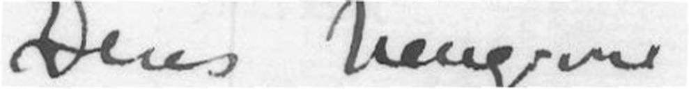Deres hengivne
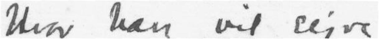Hva han vil sejre
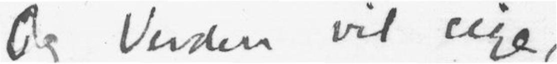Og Verden vil sige,
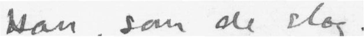Han, som de slog.
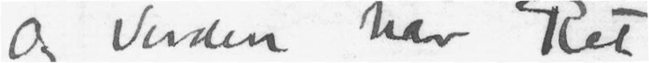Og Verden har Ret.
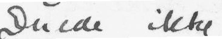Duede ikke
